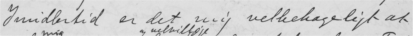Imidlertid er det mig velbehageligt at
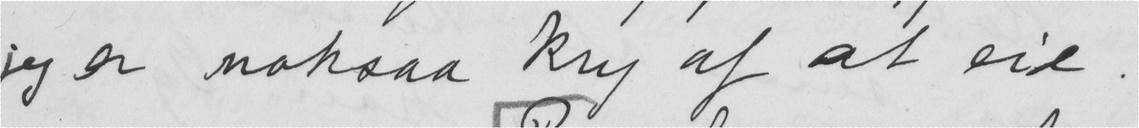jeg er noksaa krig af at eie.
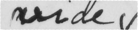vide
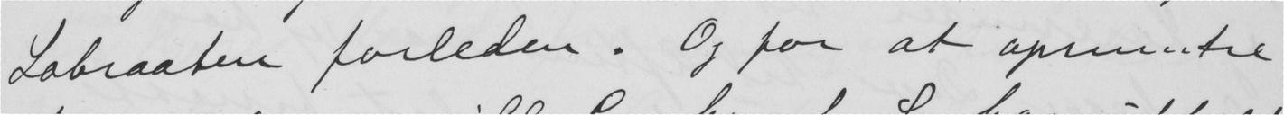Lobraaten forleden. Og for at opmuntre
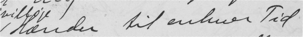Hænder til enhver Tid
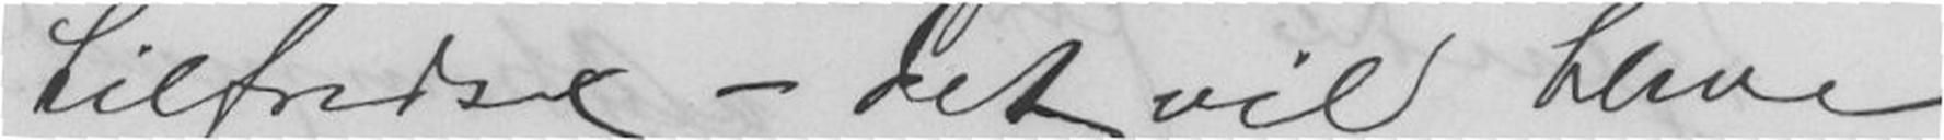tilfredse - det vil blive
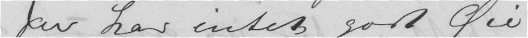Du har intet godt Øie
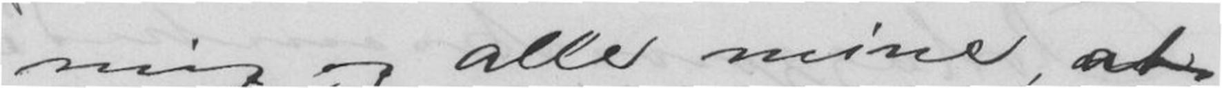mig og alle mine, at
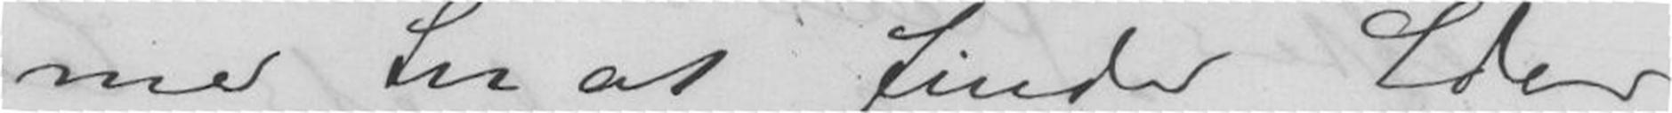me til at finde Eder
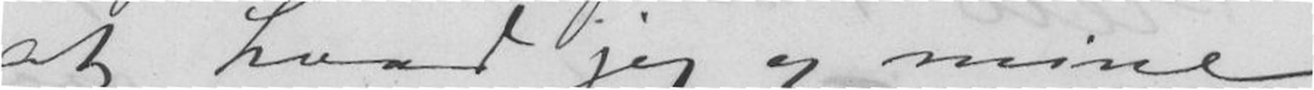at hvad jeg og mine
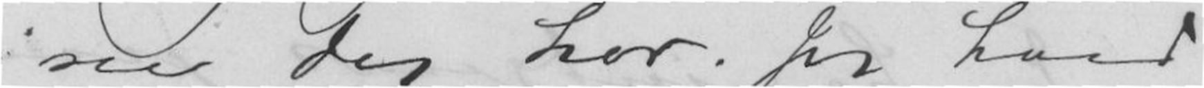see Dig her. Jeg hved
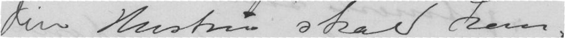din Hustru skal kom-
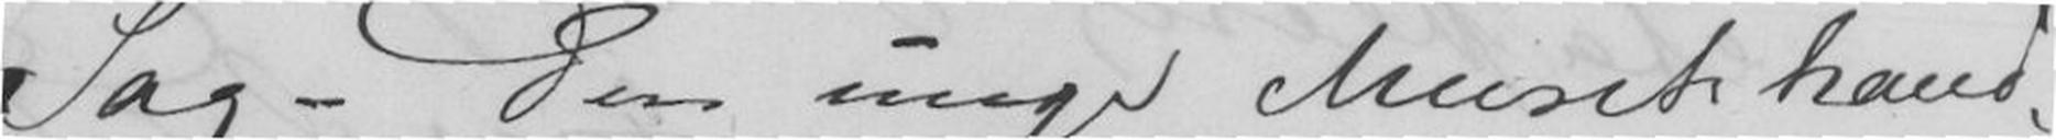Sag - Den unge Musikhand-
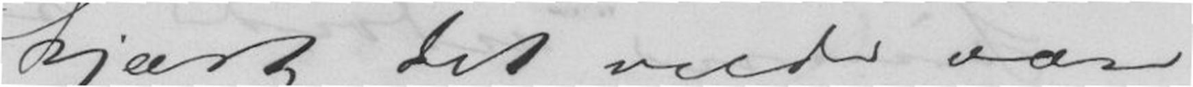kjært det vilde var
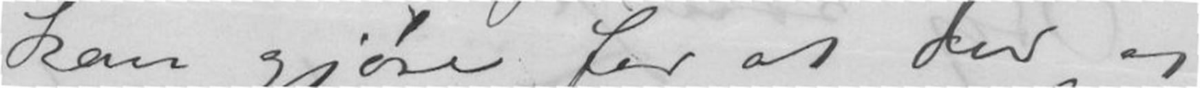kan gjøre for at du og
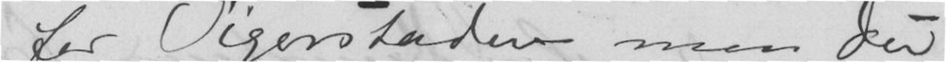for Tigerstaden men du
